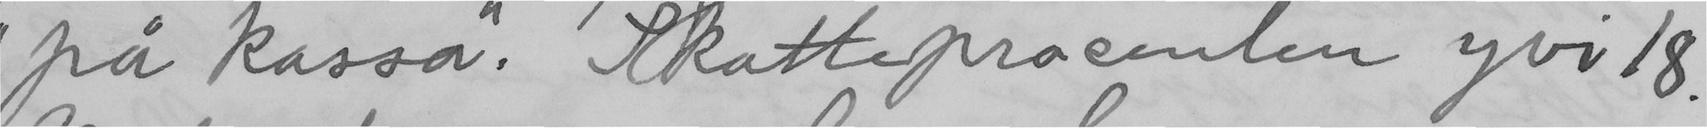"på kassa". Skatteprocenten yvi 18.
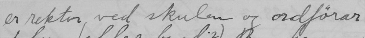er rektor ved skulen og ordförar
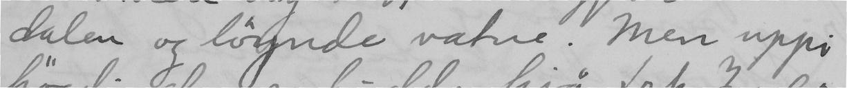dalen og løynde vatne. Men uppi
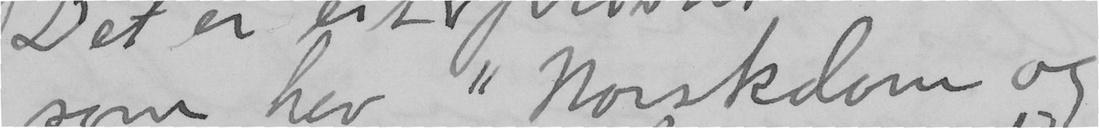som hev "Norskdom og
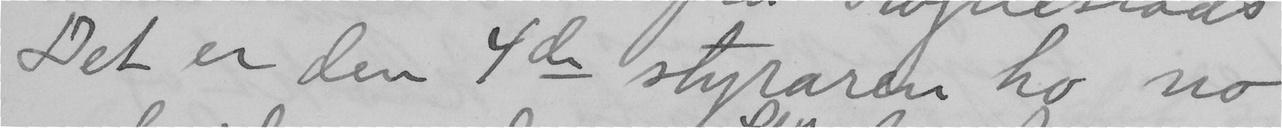Det er den 4de styraren ho no
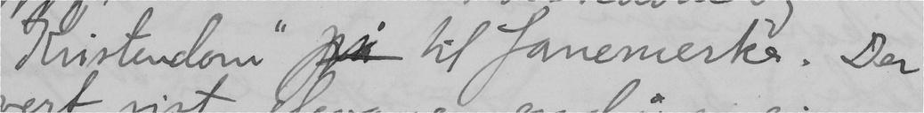Kristendom"  til fanemerke. Der
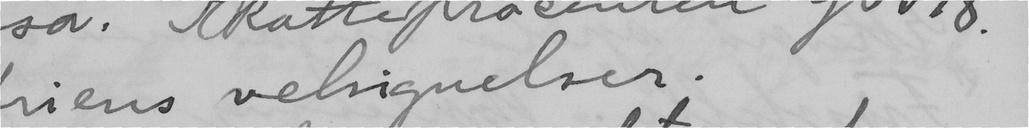Röyken frå smelteverke og
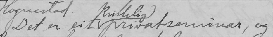Det er eit kristelig privatseminar, og
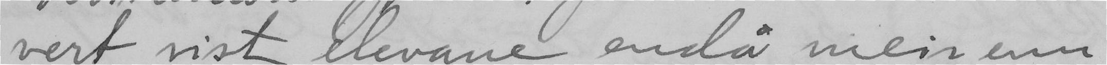vert vist elevane endå meir enn
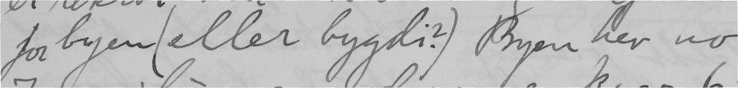for byen (eller bygdi?) Byen hev no
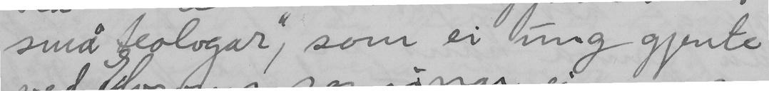små teologar", som ei ung gjente
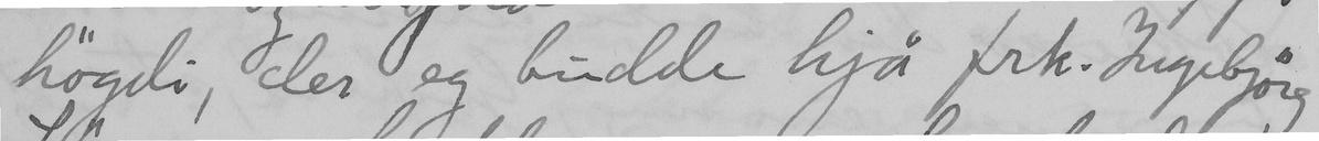högdi, der eg budde hjå frk. Ingebjörg
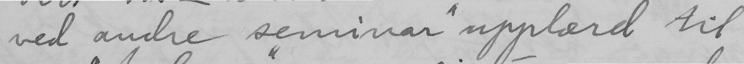ved andre seminar "upplærd til
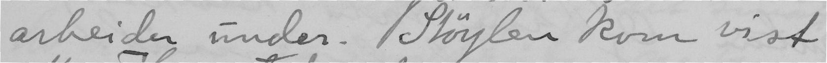arbeider under. Stöylen kom vist
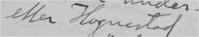etter Hognestad.
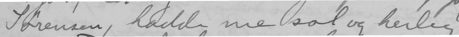Söørensen, hadde me sol og herleg
